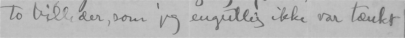to billeder, som jeg engullig ikke var tænkt
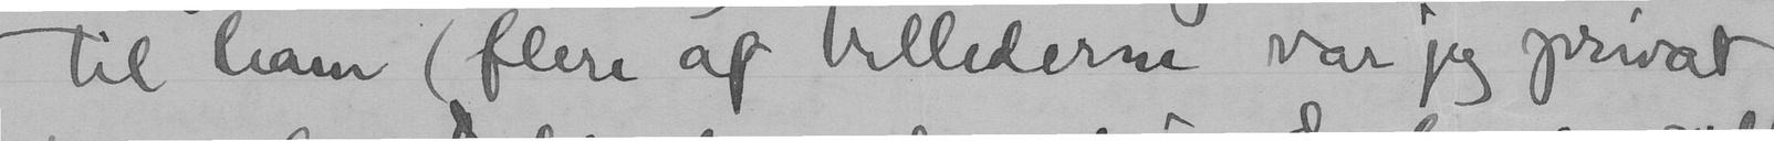til ham (flere af billederne var jeg privat
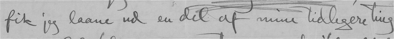fik jeg laane ud en del af mine tidligere ting
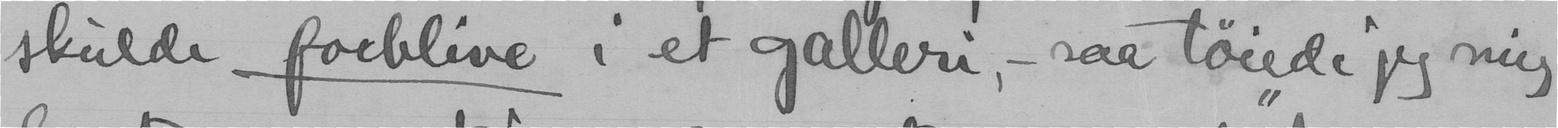skulde forblive i et galleri, - saa tøiede jeg mig
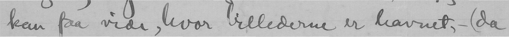kan faa vide, hvor billederne er havnet, - (da
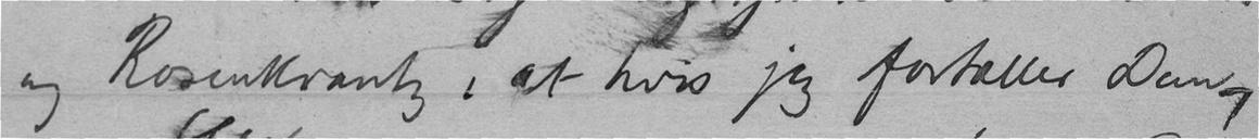og Rosenkrantz, at hvis jeg fortæller Dem,
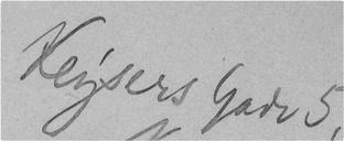Keysers Gade 5,
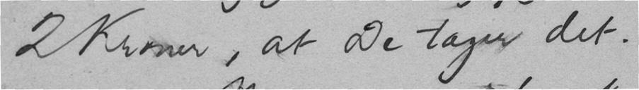2 Kroner, at De tager det.
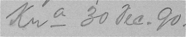Kra 30 Dec. 90.
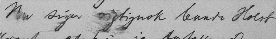Nu siger rigtignok baade Holst
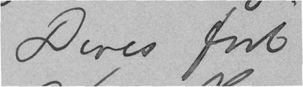Deres forb
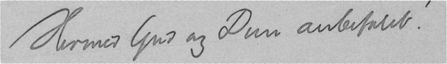Hermed Gud og Dem anbefalet!
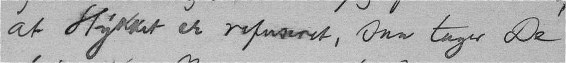at Stykket er refuseret, saa tager De
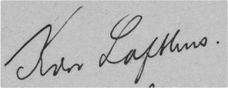Kære Lofthus.
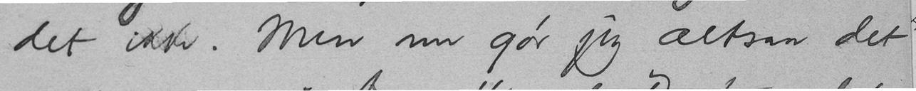det ikke. Men nu gør jeg altsaa det
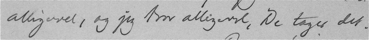alligevel, og jeg tror alligevel, De tager det.
